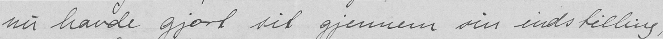nu havde gjort sit gjennem sin indstilling,
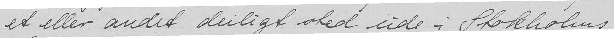et eller andet deiligt sted ude i Stokholms
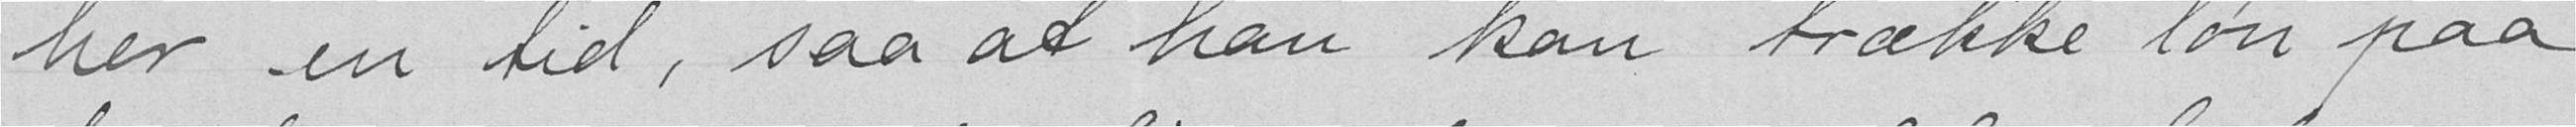her en tid, saa at han kan trække løn paa
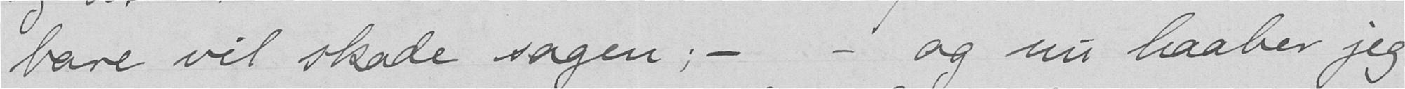bare vil skade sagen,- - og nu haaber jeg
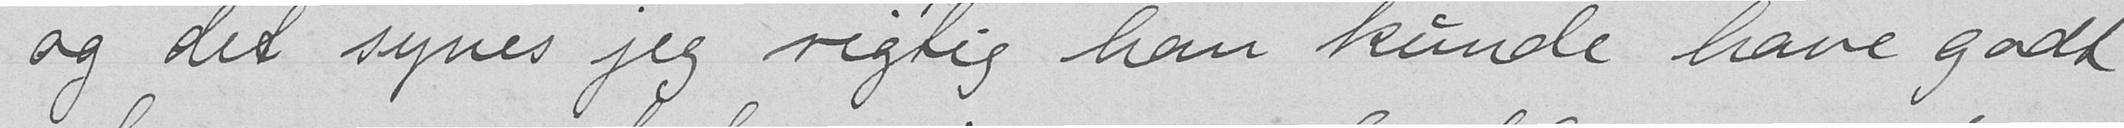og det synes jeg rigtig han kunde have godt
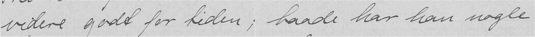videre godt for tiden; baade har han nogle
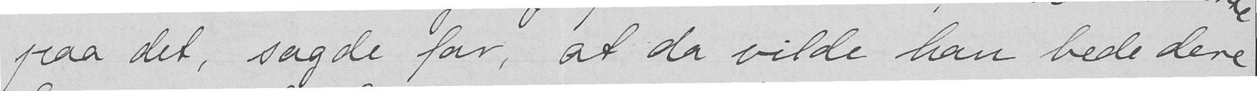paa det, sagde far, at da vilde han bede dere
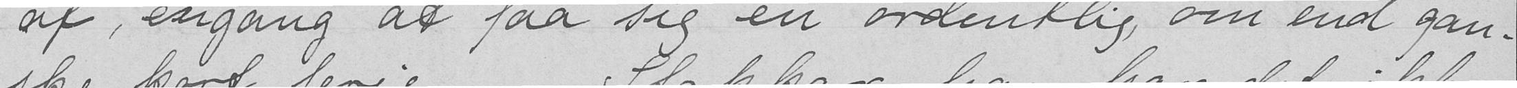af, engang at faa sig en ordentlig, om end gan-
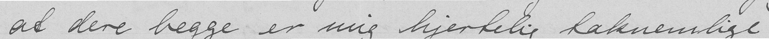at dere begge er mig hjertelig taknemlige
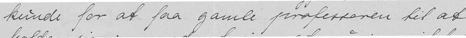kunde for at faa gamle professoren til at
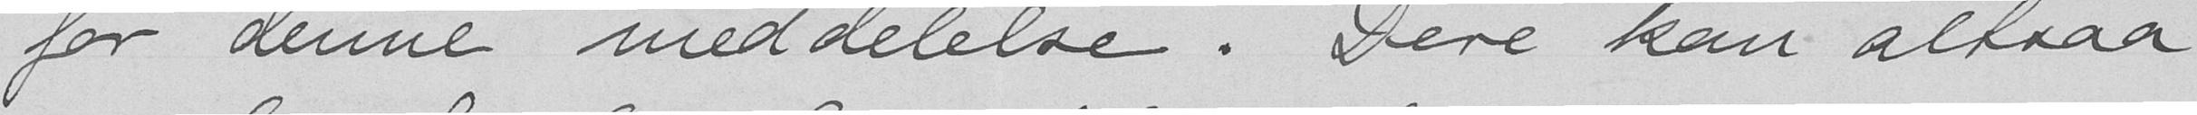for denne meddelelse. Dere kan altsaa
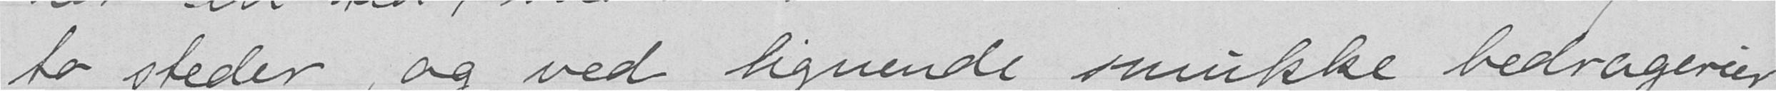to steder, og ved lignende smukke bedragerier
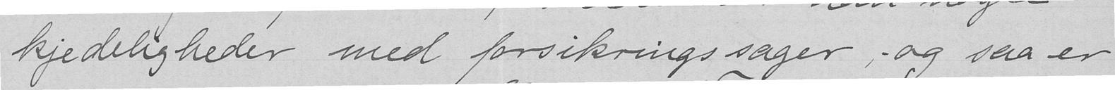kjedeligheder med forsikringssager, - og saa er
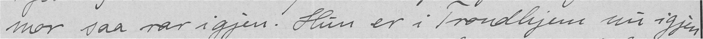mor saa rar igjen. Hun er i Trondhjem nu igjen
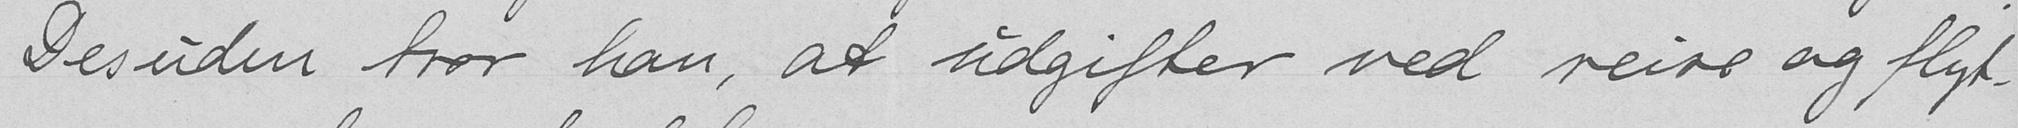Desuden tror han at udgifter ved reise og flyt-
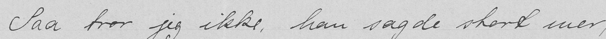Saa tror jeg ikke, han sagde stort mer,
